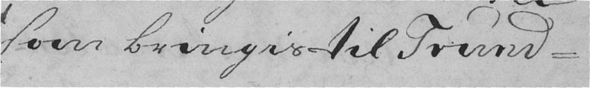som bringes til Trund-
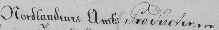Nordlandenis Amts Producter, ere
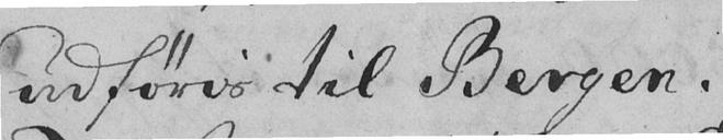udføris til Bergen.
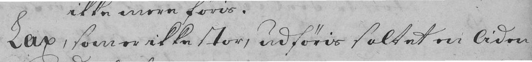Lax, som er ikke stor, udføris saltet en liden
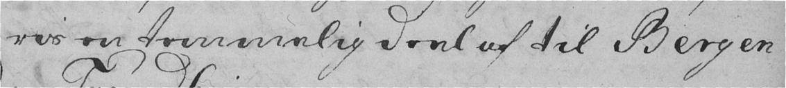ris en temmelig deel af til Bergen
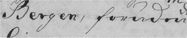Bergen, foruden
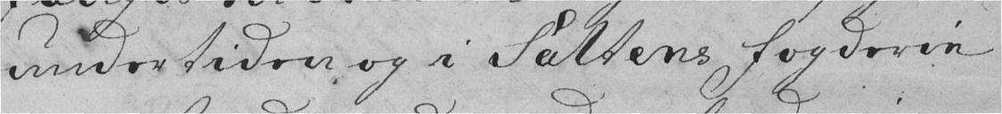undertiden og i Saltens Fogderie
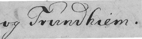og Trundhiem.
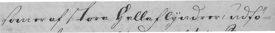som er af store helleflyndrer, udfø-
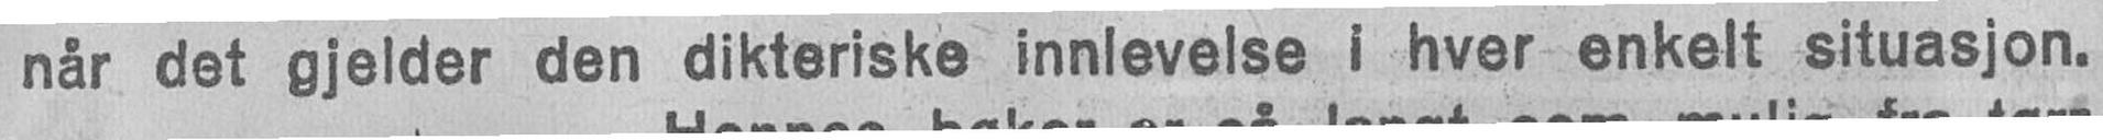når det gjelder den dikteriske innlevelse i hver enkelt situasjon.
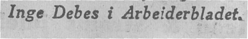Inge Debes i Arbeiderbladet.
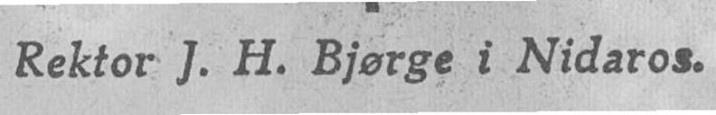Rektor J. H. Bjørge i Nidaros.
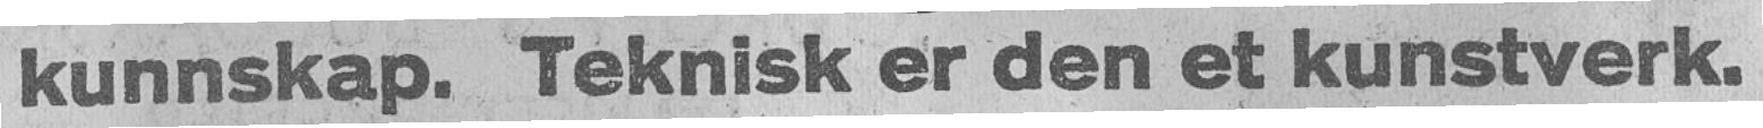kunnskap. Teknisk er den et kunstverk.
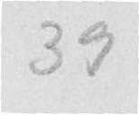39
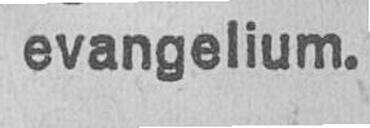evangelium.
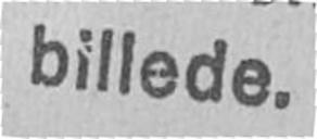billede.
